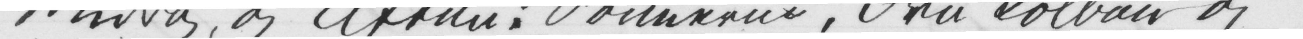Middag og Aften: Sønnerne, Fru Colban og
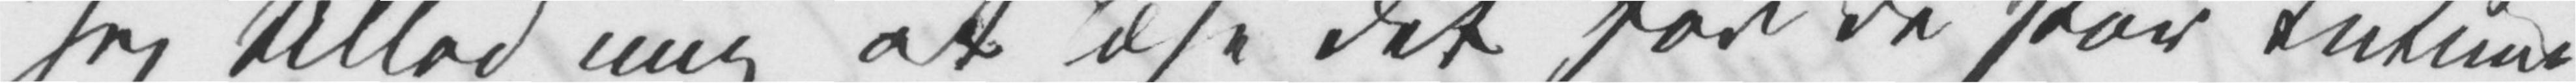Jeg tillod mig at læse det for de Par Intime
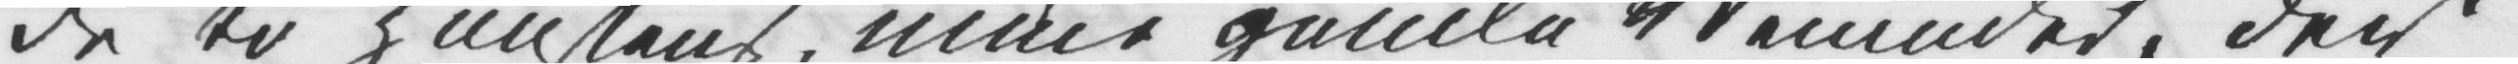de to Hanstens, mine gamle Veninder, der
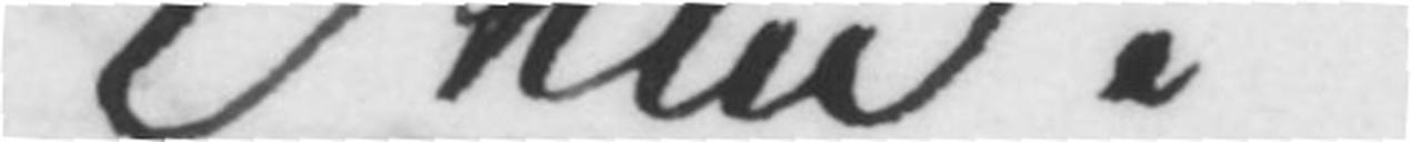Dem.
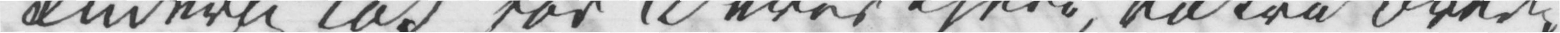Inderlig Tak for Deres kjere, vakre Brev.
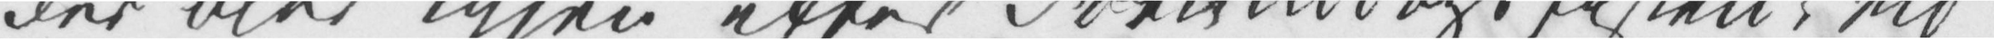der blev igjen efter Formiddagsfesten, til
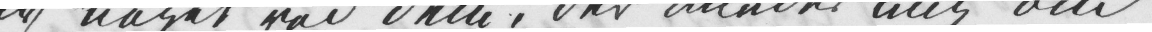er noget ved dem, der minder mig om
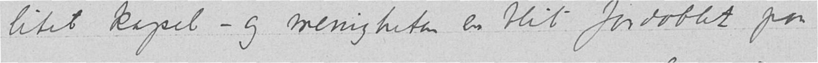litet kapel – og menigheten er blit fordoblet paa
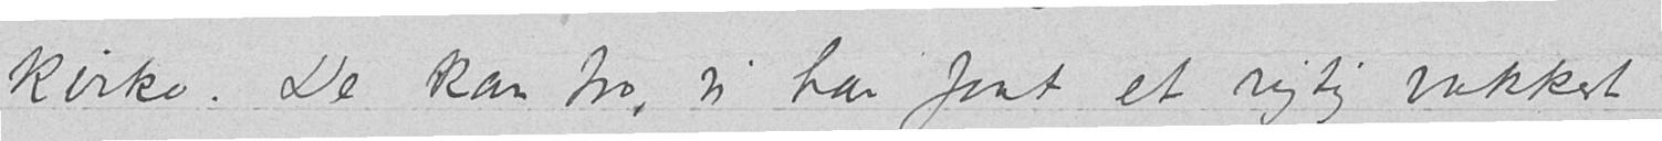kirke. De kan tro, vi har faat et rigtig vakkert
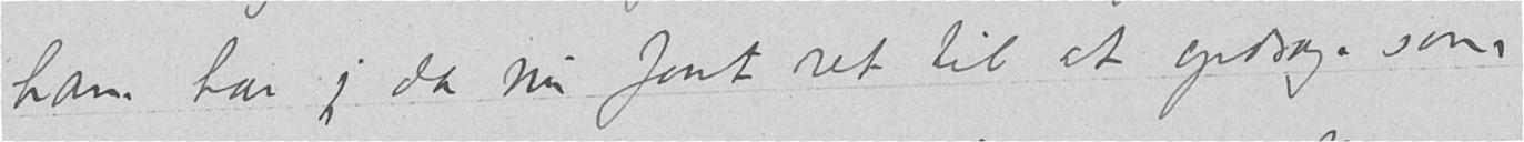ham har jeg da nu faat ret til at opdrage som
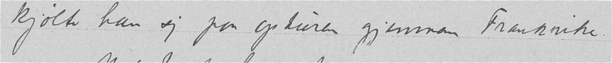kjølte han sig paa opturen gjennem Frankrike.
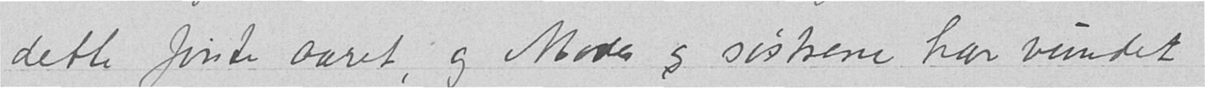dette første aaret, og Moder og søstrene har vundet
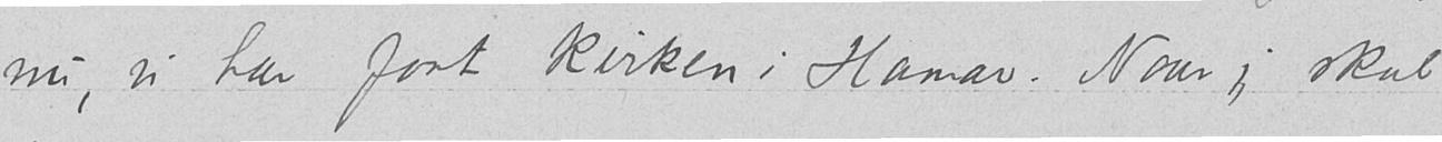nu, vi har faat kirken i Hamar. Naar jeg skal
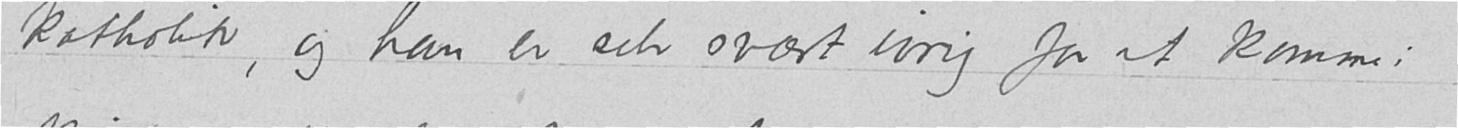katholik, og han er selv svært ivrig for at komme i
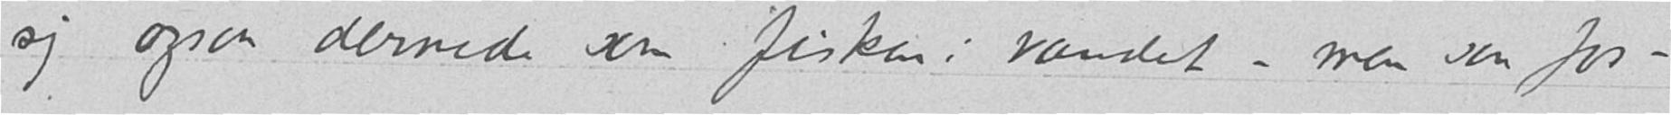sig ogsaa dernede som fisken i vandet – men saa for-
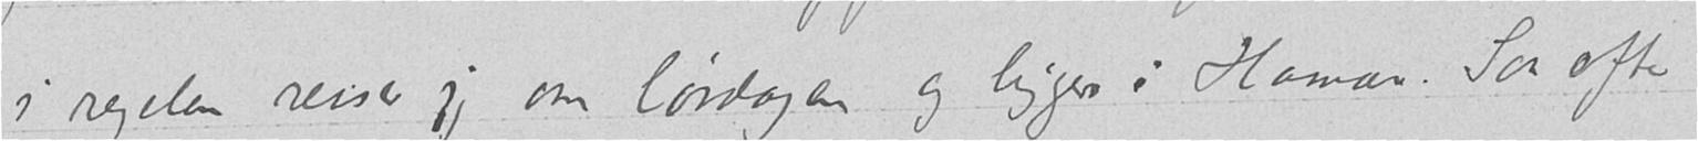i regelen reiser jeg om lørdagen og ligger i Hamar. Saa ofte
